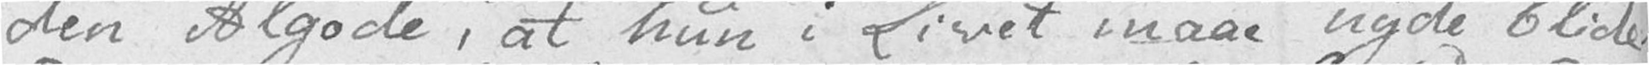den Algode, at hun i Livet maae nyde blide
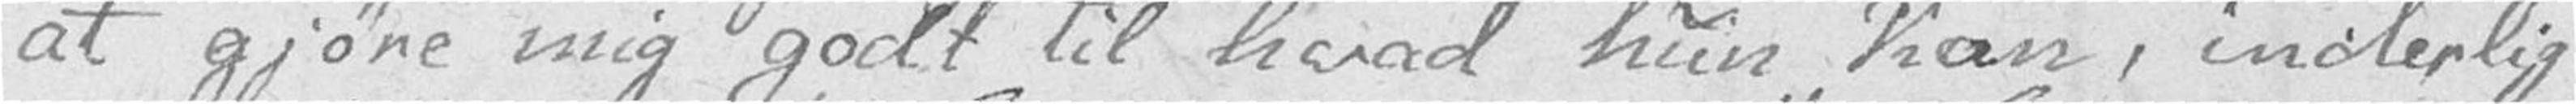at gjøre mig godt til hvad hun kan, inderlig
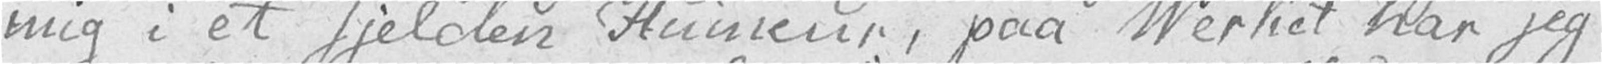mig i et sjelden Humeur, paa Verket har jeg
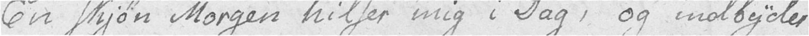En skjøn Morgen hilser mig i Dag, og indbyder
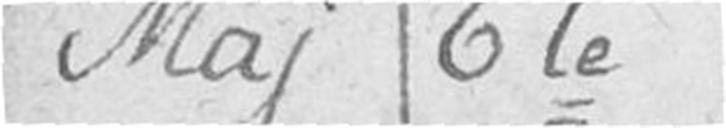Maj 6te
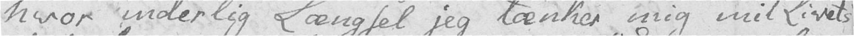hvor inderlig Længsel jeg tænker mig mit Livets
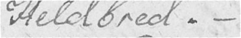Heldbred. –
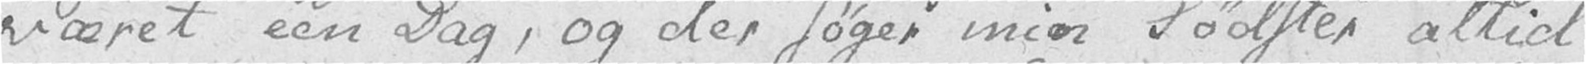været een Dag, og der søger min Sødster altid
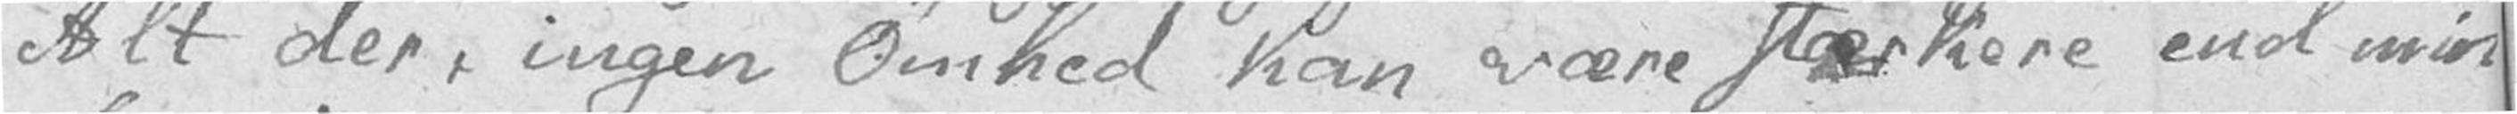Alt der, ingen Ømhed kan være stærkere end min
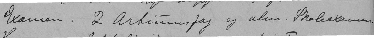Exmen. 2 Artiumsfag og alm. Skoleexamen.
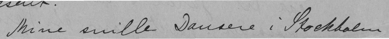Mine snille Dansere i Stockholm
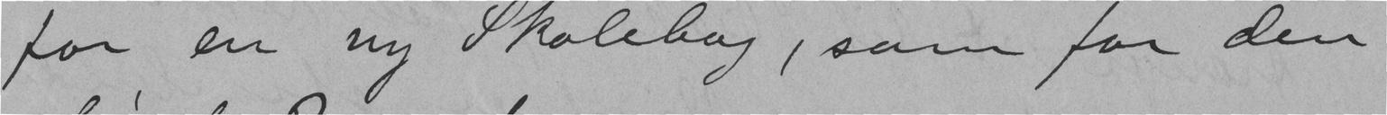for en ny Skolebog, som for den
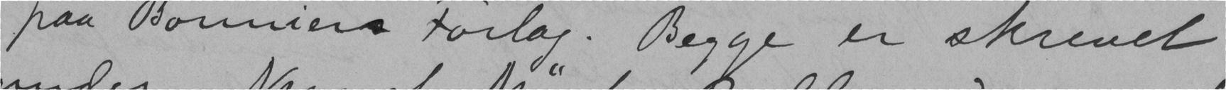paa Bonniers Forlag. Begge er skrevet
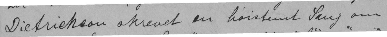Dietrickson skrevet en høistemt Sang om
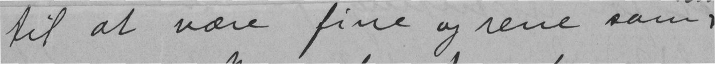til at være fine og rene som
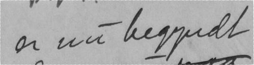er nu begyndt
